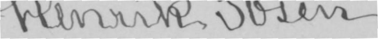Henrik Ibsen.
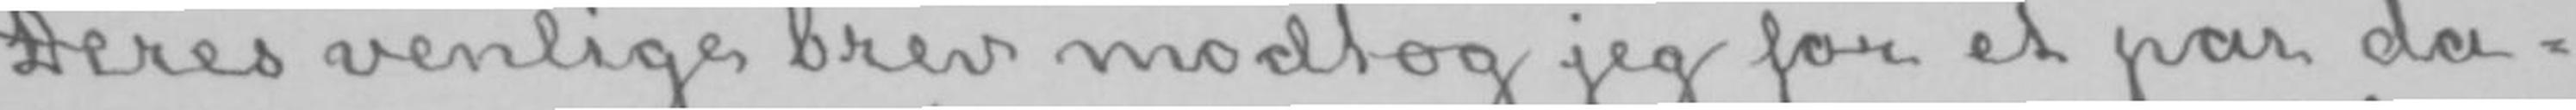Deres venlige brev modtog jeg for et par da-
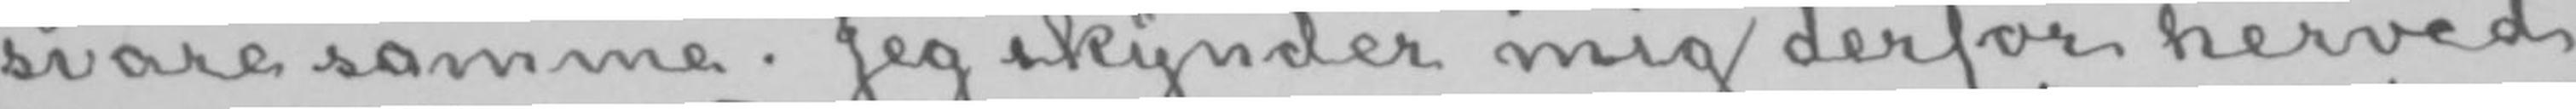svare samme. Jeg skynder mig derfor herved
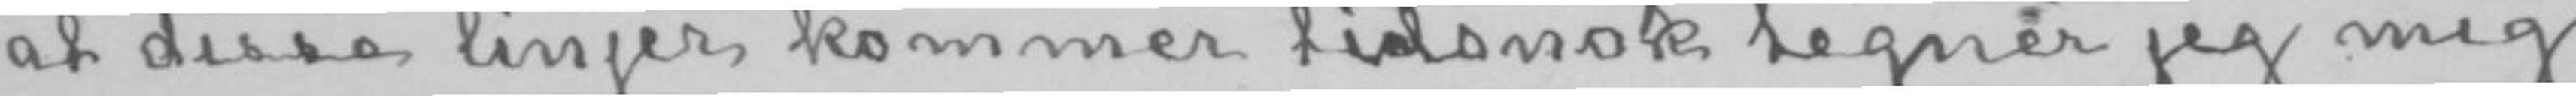at disse linjer kommer tidsnok tegner jeg mig
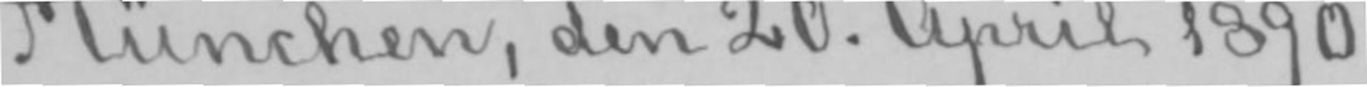München, den 20. April 1890
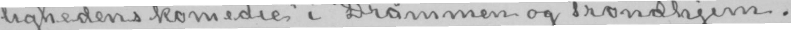lighedens komedie» i Drammen og Trondhjem.
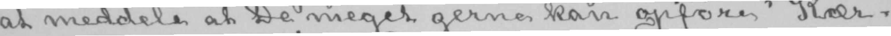at meddele at De meget gerne kan opføre «Kær-
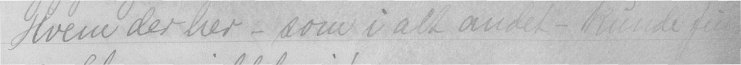Hvem der her - som i alt andet - kunde finde
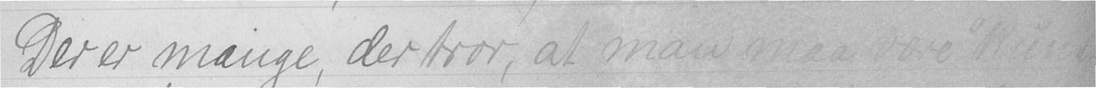Der er mange, der tror, at man maa være "kunst-
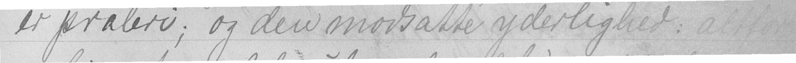er praleri; og den modsatte yderlighed: altfor tar-
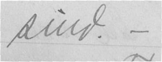sind. -
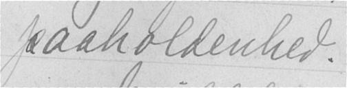paaholdenhed.
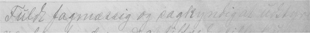Fuldt fagmæssig og sagkyndig at udstyre
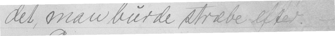det, man burde stræbe efter. -
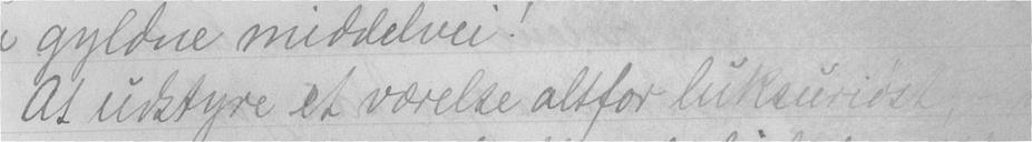At udstyre et værelse altfor luksuriøst,
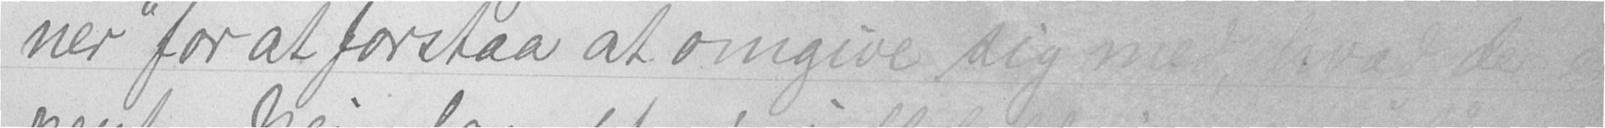ner" for at forstaa at omgive sig med, hvad der er
